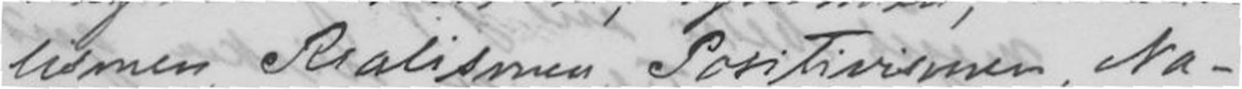lismen, Realismen, Positivismen, Na-
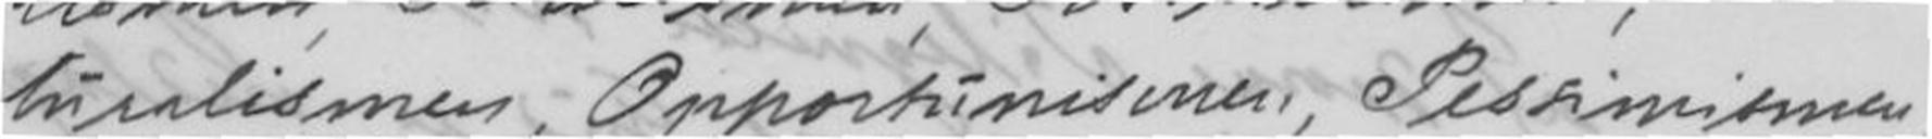turalismen, Opportunismen, Pessimismen,
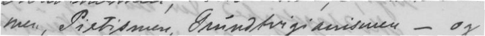men, Pietismen, Grundtvigianismen - og
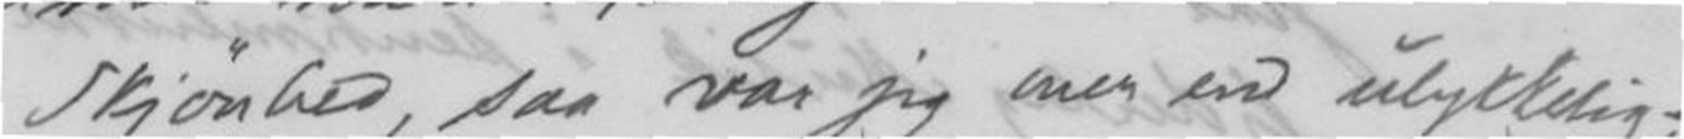Skjønhed, saa var jeg mer end ulykkelig;.
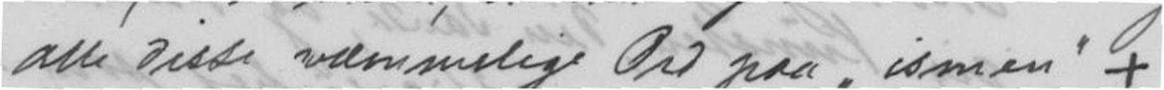alle disse væmmelige Ord paa ismen +
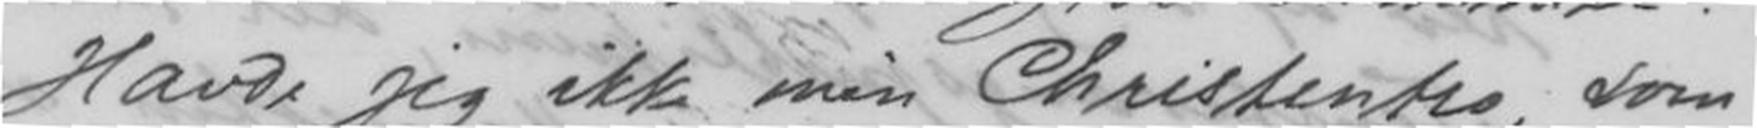Havde jeg ikke min Christentro, som
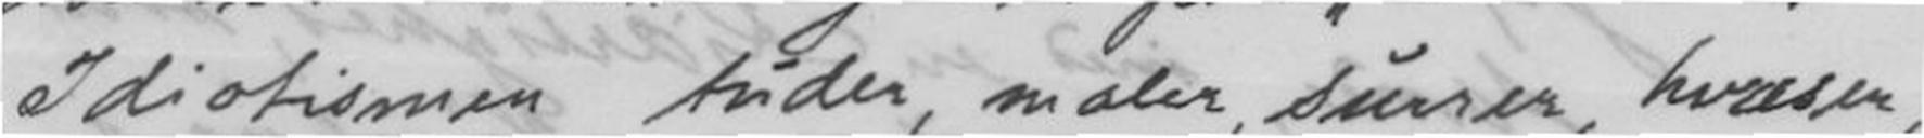Idiotismen tuder, maler, surrer, hvaser,
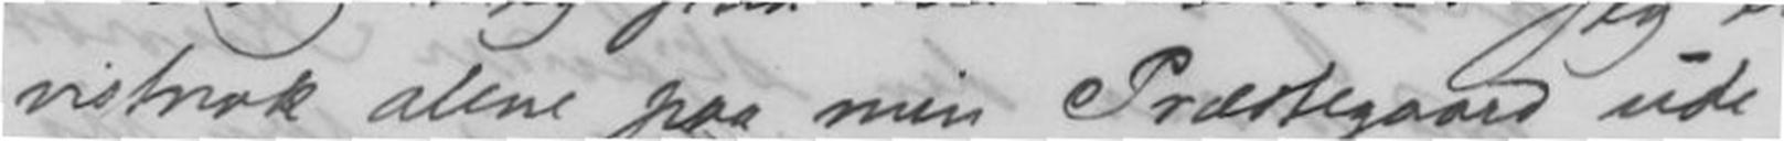vistnok alene paa min Præstegaard ude
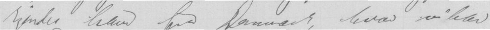kjender ham fra Danmark, hvor vi har
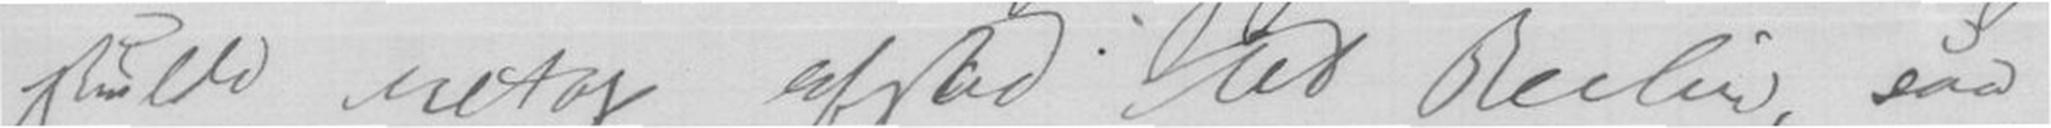skulde netop afsted til Berlin, saa
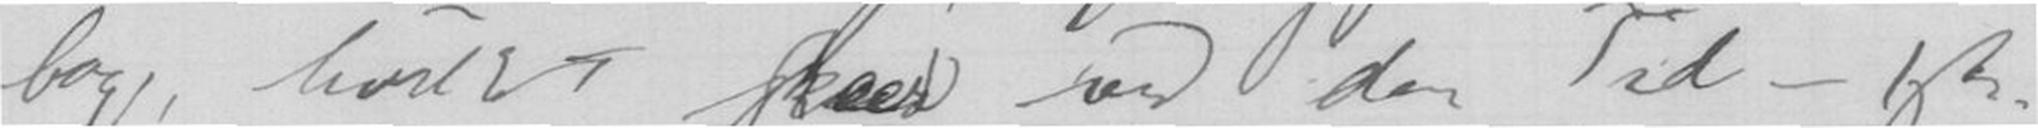bage, hvilket skeer ved den Tid - 1ste
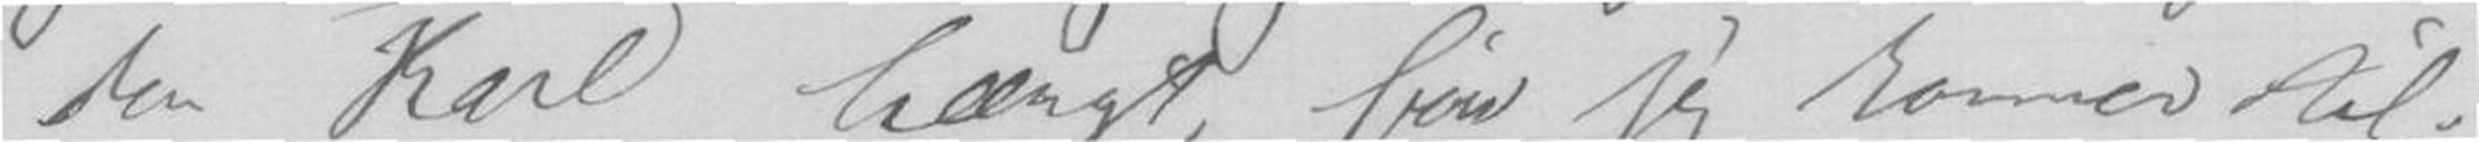den Karl hængt, før jeg kommer til-
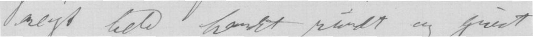reist hele landet rundt og givet
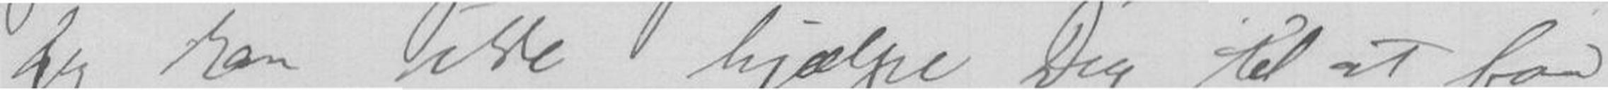jeg kan ikke hjælpe Dig til at faa
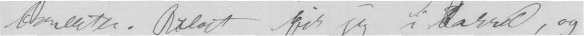Concerter. Brevet fik jeg i Cassel, og
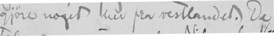gjøre noget her fra vestlandet. De
